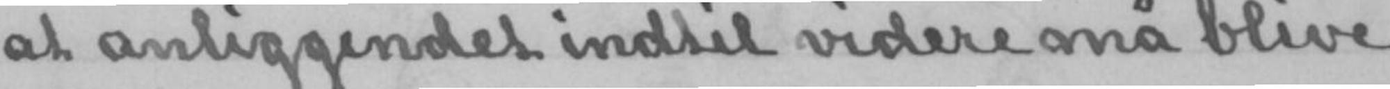at anliggendet indtil videre må blive
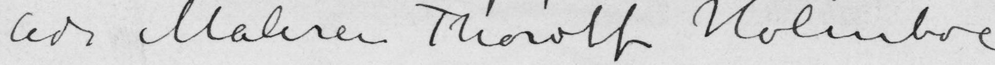Adr Maleren Thorolf Holmboe
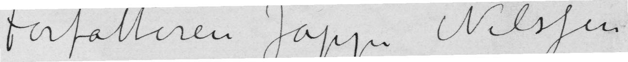Forfatteren Jappe Nilssen
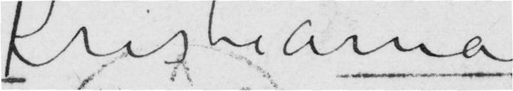Kristiania
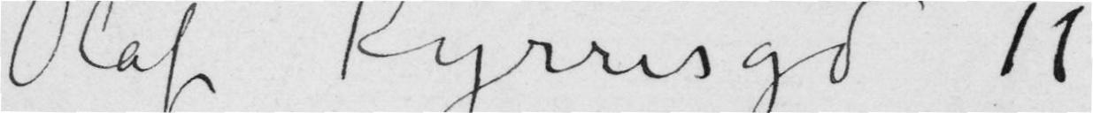Olaf Kyrresgd 11
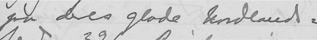paa deres glade Nordlands-
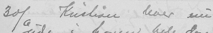30/6 Kristian lever nu
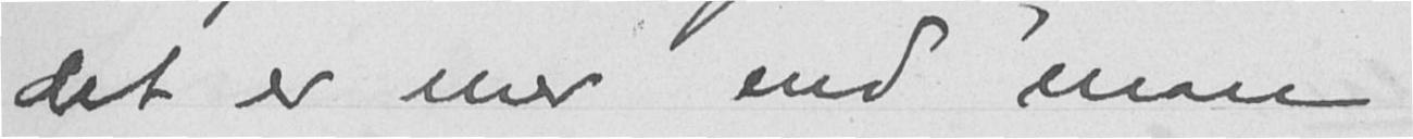det er mer end man
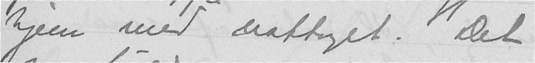hjem med nattoget. Det
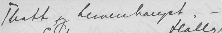Thott og Lewenhaupt, -
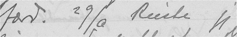færd. 29/6 Reiste jeg
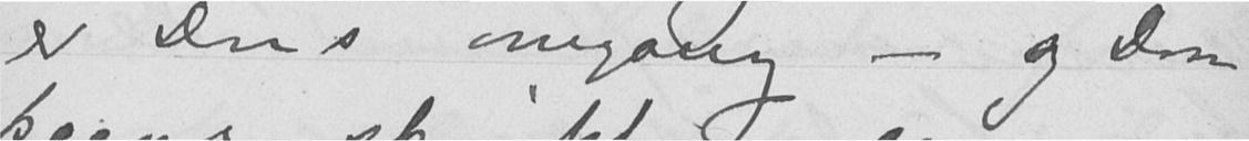er Dons omgang - og Don
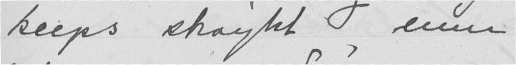keeps straight men
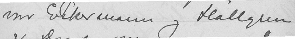var Eckermann og Hallgren
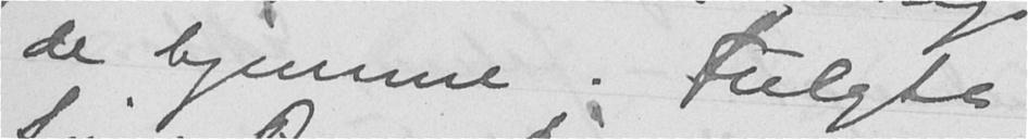de hjemme. Fulgte
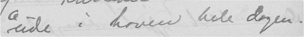ude i haven hele dagen.
